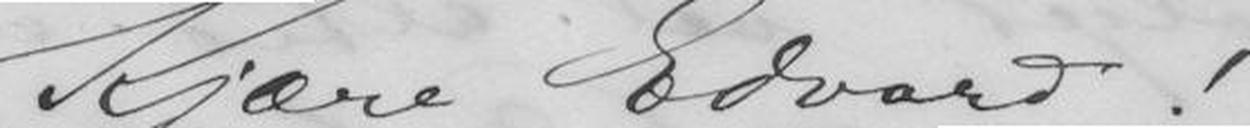Kjære Edvard!
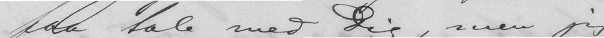faa tale med Dig, men jeg
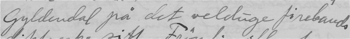Gyldendal på det velduge firebands
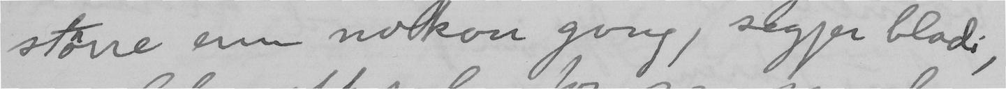större enn nokon gang, segjer bladi,
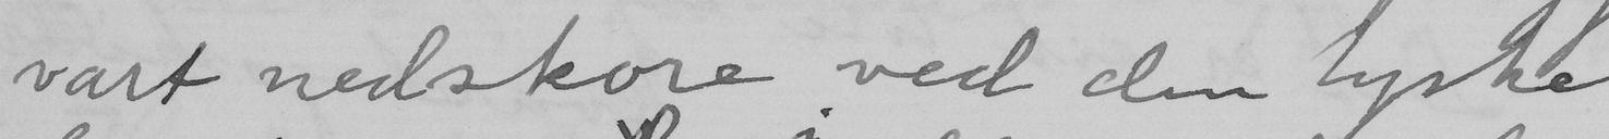vart nedskore ved den tyske
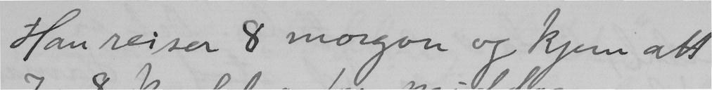Han reiser 8 morgon og kjem att
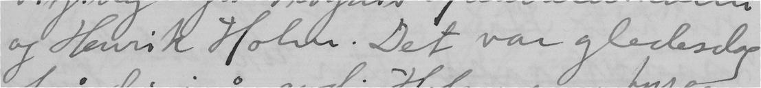og Henrik Holm. Det var gledesdag
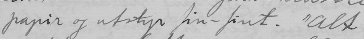papir og utstyr fin-fint. "Alt
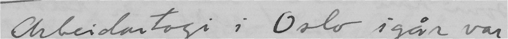Arbeidartogi i Oslo igår var
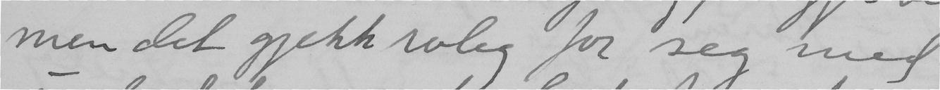men det gjekk roleg for seg med
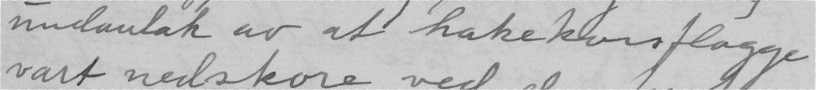undantak av at hakekorsflagge
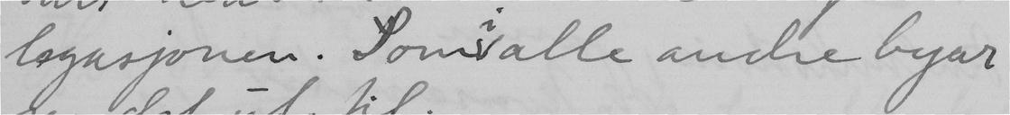legasjonen. Som i alle andre byar
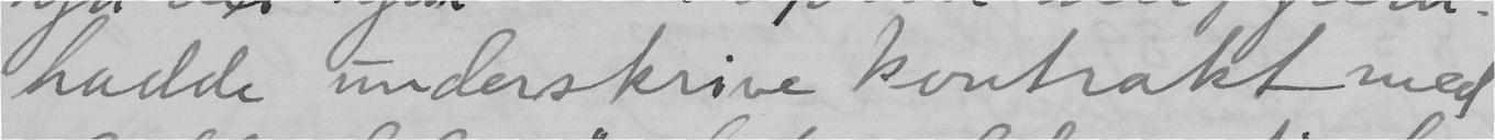hadde underskrive kontrakt med
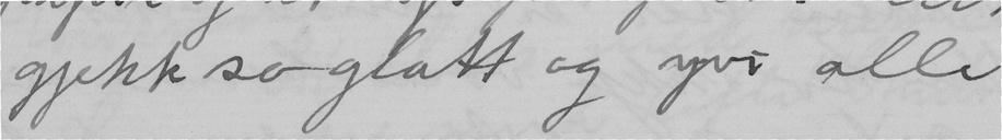gjekk so glatt og yvi alle
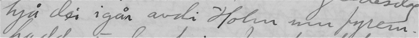hjå dei igår avdi Holm um fyrern.
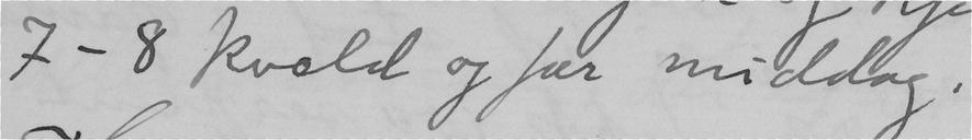7-8 kveld og fær middag.
